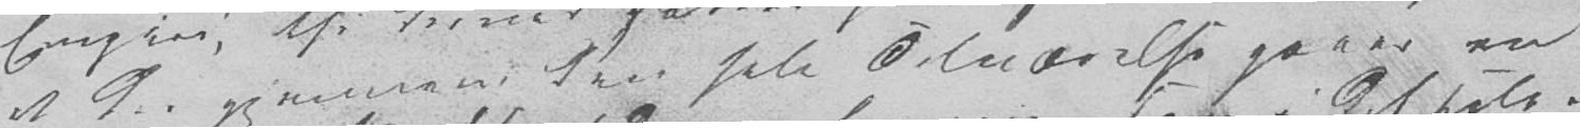at der gjennem den hele Tilværelse gaaer en
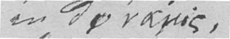en Doraxis,
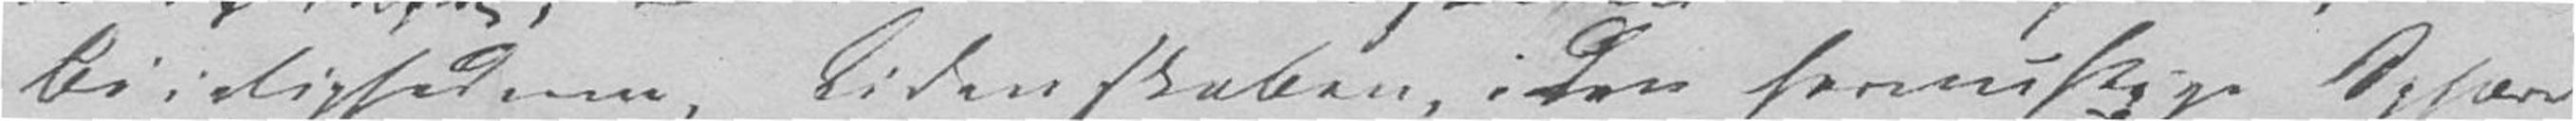bøielighederne, Lidenskaben, i den fornuftige Sphære
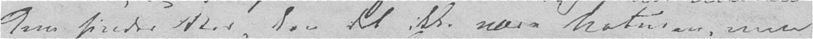dem finder Sted, kan det ikke være Naturen, men
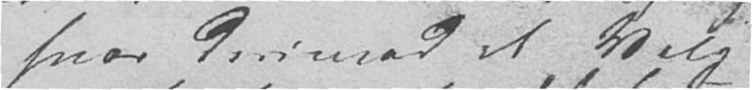hvor derimod et Valg
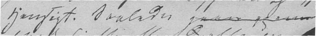Hensigt. Saaledes
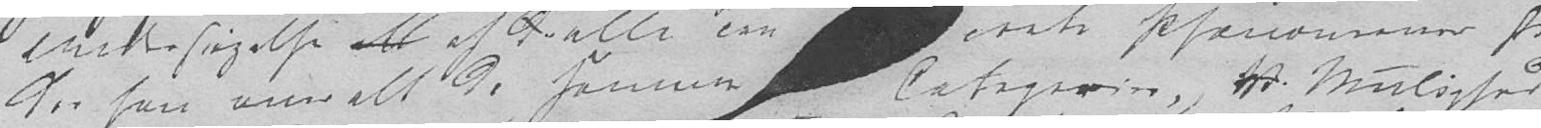der han overalt de samme Categorier. V Mulighed
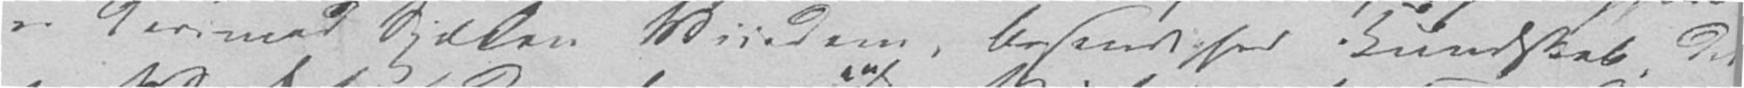er derimod Sjælen Viisdom, Besindighed, Kundskab. Der
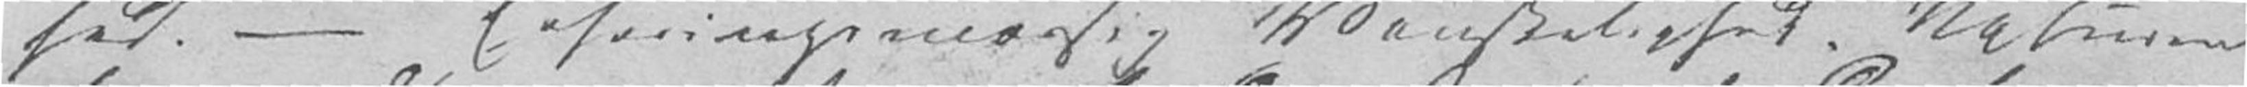hed. – Erfaringsmæssig Vanskelighed. Naturen
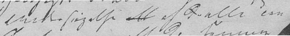Undersøgelse all af de alle con-
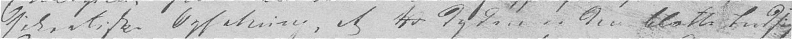sokratiske Opfatning, at V Dyden er den blotte Indsigt,
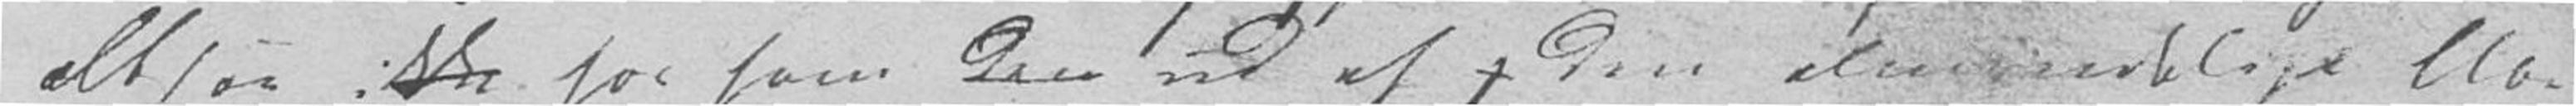altsaa ikke hos ham den ud af den almindelige Ela-
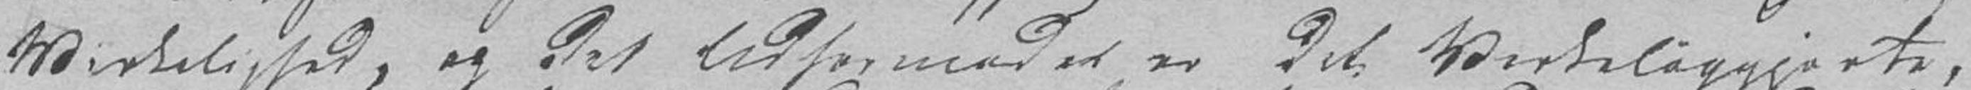Virkelighed, og det Udformede er det Virkeliggjorte,
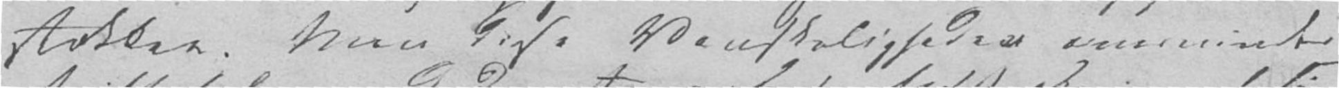stokken. Men disse Vanskeligheder overvinder
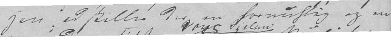Han adskiller der en fornuftig og en
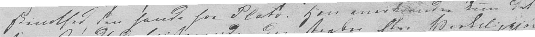stiskhed den havde hos Plato. Han anerkjender kun det
